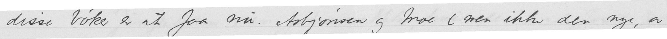disse bøker er at faa nu. Asbjønsen og Moe (men ikke den nye, av
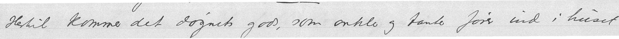Hertil kommer det døgnets gods, som onkler og tanter fører ind i huset
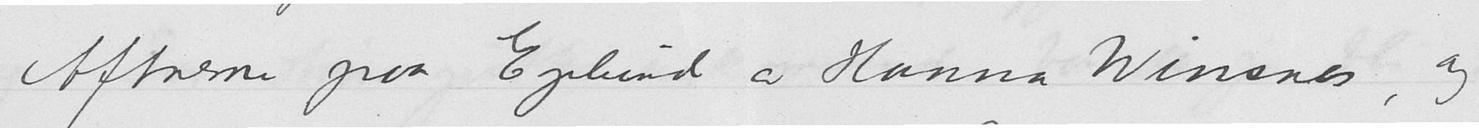Aftnerne paa Egelund av Hanna Winsnes, og
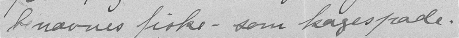benævnes fiske- som kagespade.
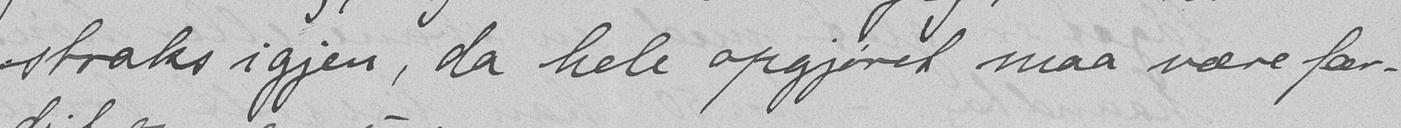straks igjen, da hele opgjøret maa være fær-
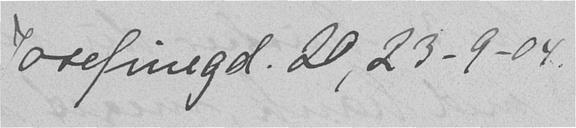Josefinegd. 20, 23-9-04.
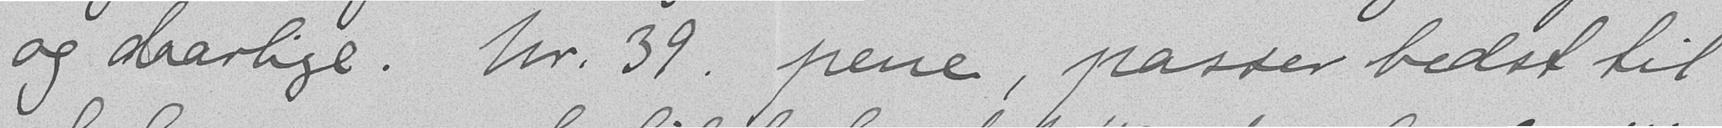og daarlige. Nr. 39. pene, passer bedst til
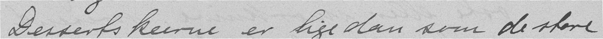Dessertskeerne er ligedan som de store
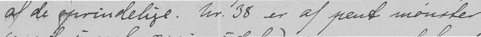af de oprindelige. Nr. 38. er af pent mønster
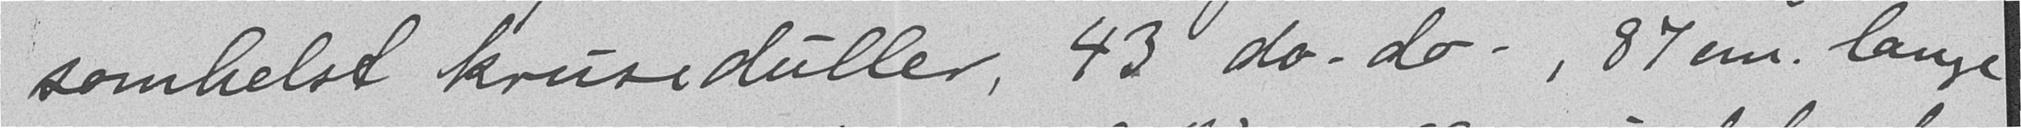somhelst kruseduller, 43 do-do - , 87 cm. lange
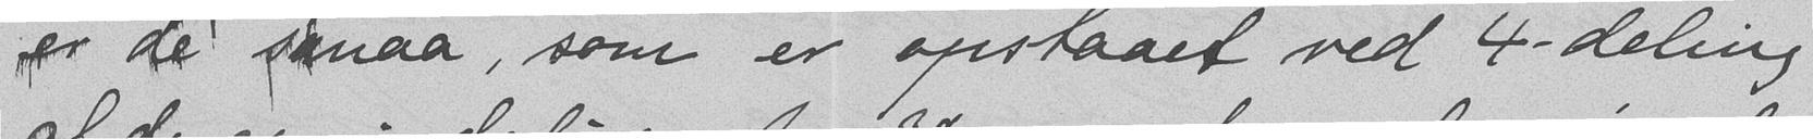er de smaa, som er opstaaet ved 4-deling
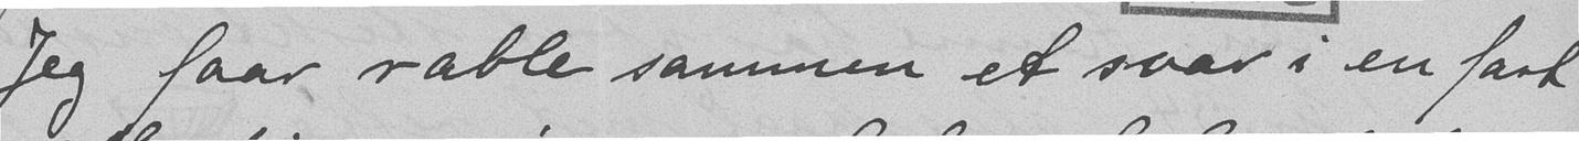Jeg faar rable sammen et svar i en fart
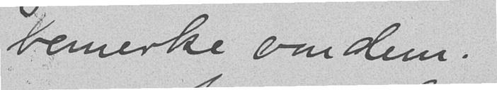bemerke om dem.
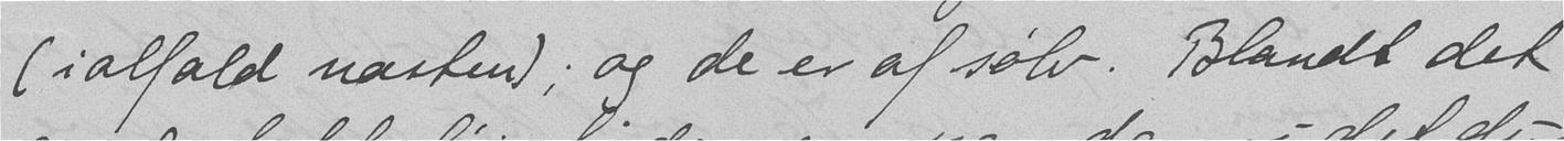(ialfald næsten); og de er af sølv. Blandt det
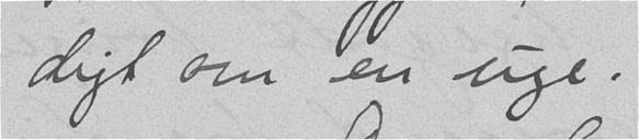digt om en uge.
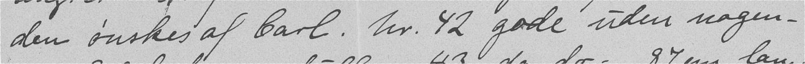den ønskes af Carl. Nr. 42 gode uden nogen-
